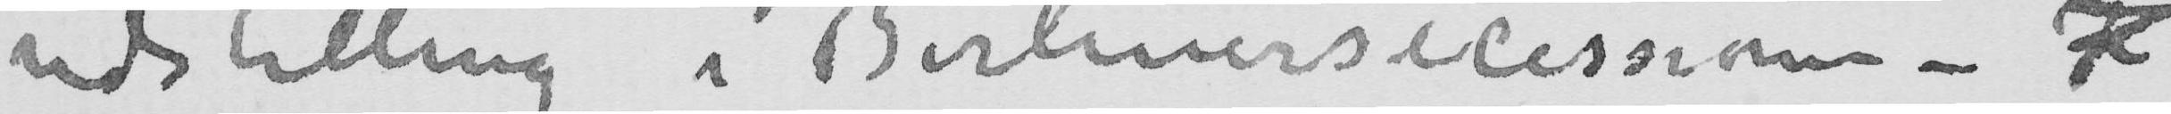udstilling i Berlinersecessionen –
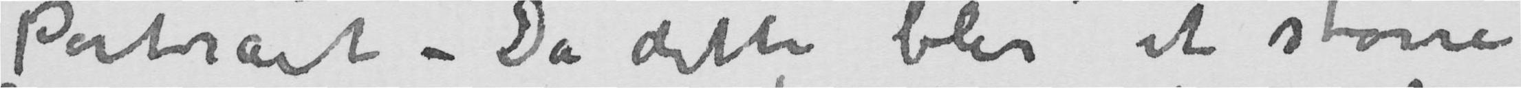Portrait – Da dette blir et større
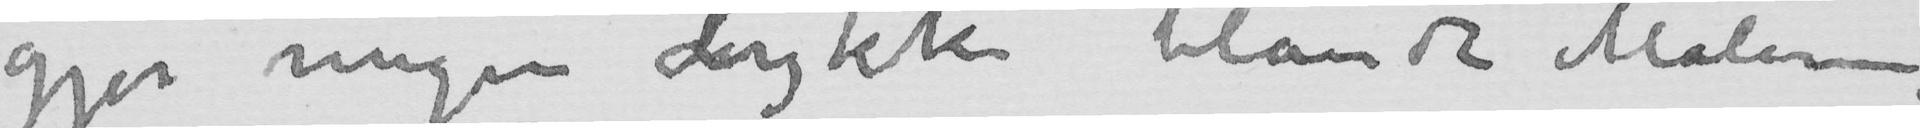gjør megen Lykke blandt Malerne
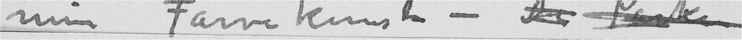min Farvekunst – D Parken
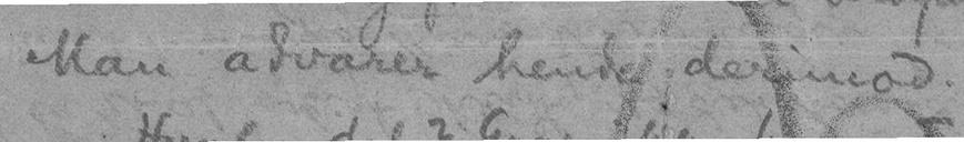Man advarer hende derimod.
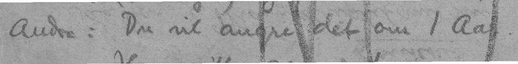Andre: Du vil angre det om 1 Aar.
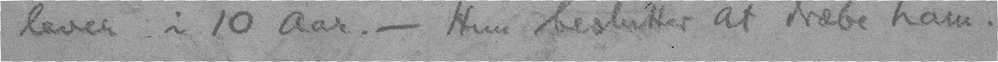lever i 10 Aar. - Hun beslutter at dræbe ham.
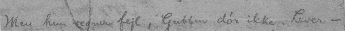Men hun regner fejl, Gubben dør ikke. Lever -
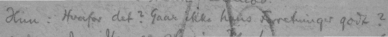Hun: Hvorfor det? Gaar ikke hans Forretninger godt?
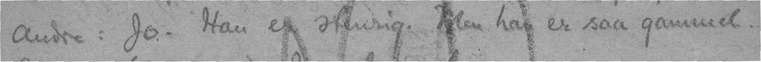Andre: Jo. Han er stenrig. Men han er saa gammel.
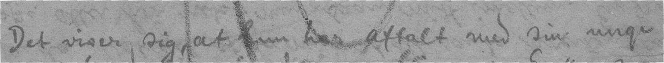Det viser sig at hun har aftalt med sin unge
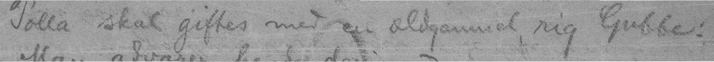Polla skal giftes med en ældgammel rig Gubbe.
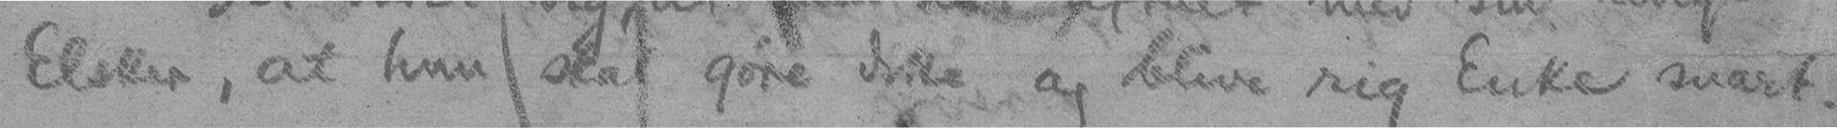Elsker, at hun skal gøre dette og blive rig Enke snart.
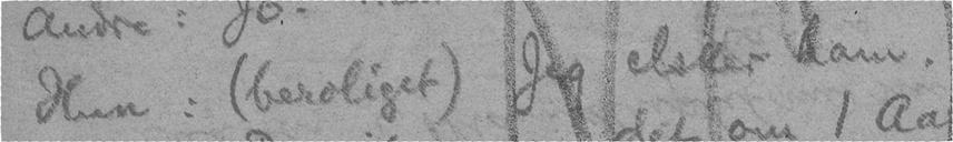Hun: (beroliget) Jeg elsker ham.
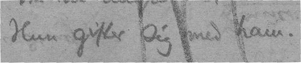Hun gifter sig med ham.
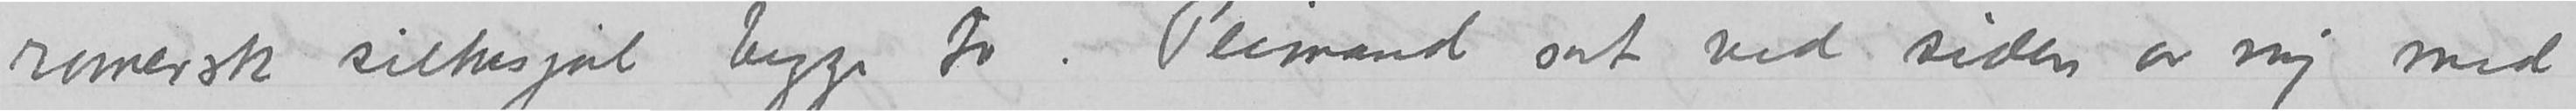romersk silkesjal begge to. Peimand sat ved siden av mig med
